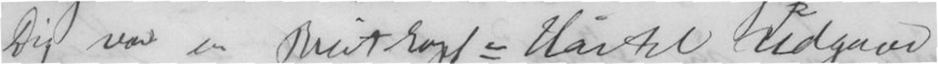Dig var en Breitkopf=Härtel Udgaven,
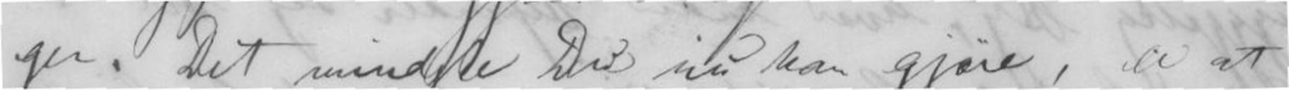ger. Det mindste Du nu kan gjøre, er at
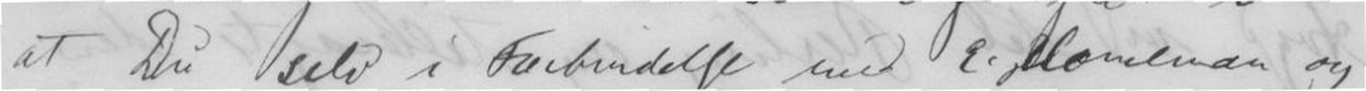at Du selv i Forbindelse med E. Horneman og
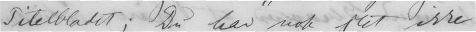Titelbladet; Du har nok slet ikke
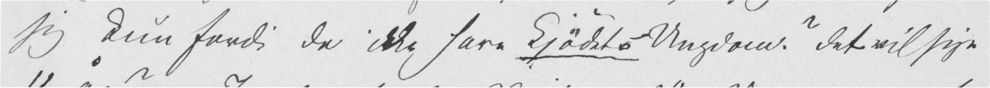seg kun fordi de ikke have Kjødets Ungdom? Det vil sige
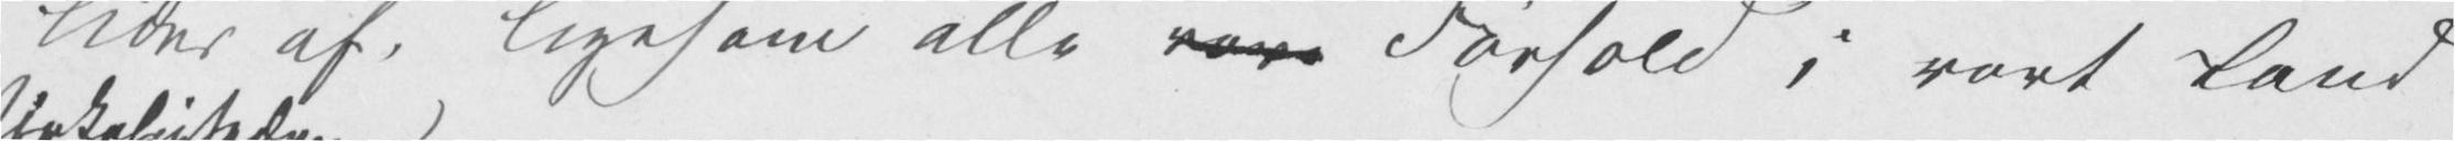lider af, ligesom alle vore Forhold i vort Land
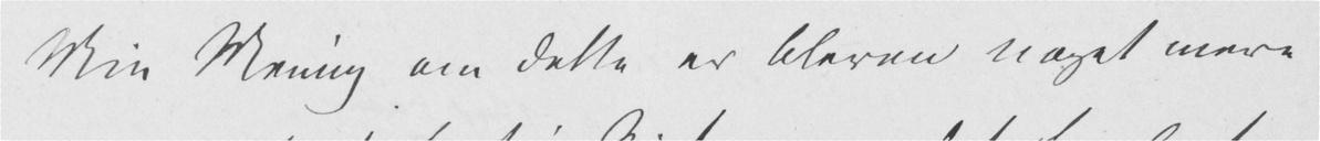Min Menig om dette er bleven noget mere
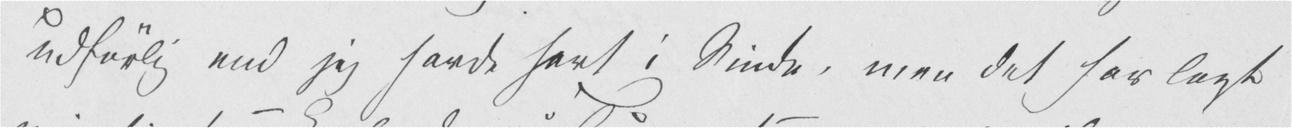udførlig end jeg havde havt i Sinde, men det har lagt
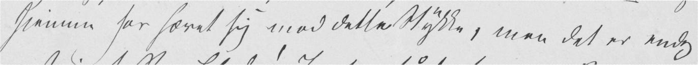hjemme har hævet sig mod dette Stykke, men det er endog
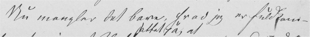Nu mangler det bare, hvad jeg er fuldkom-
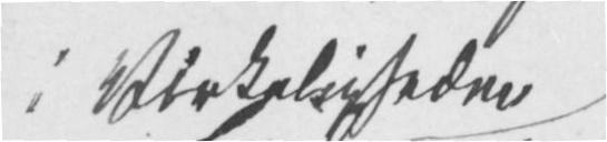i Virkeligheden
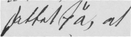fattet på, at
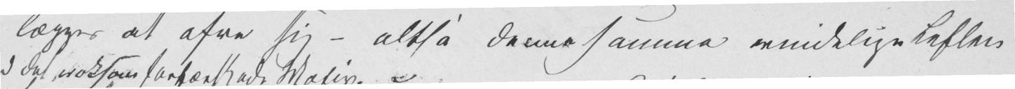lægges at ofre sig – altså denne samme evindelige Leflen
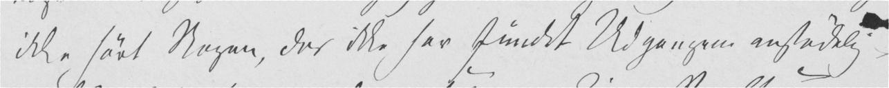ikke hørt Nogen, der ikke har fundet Udgangen anstødelige
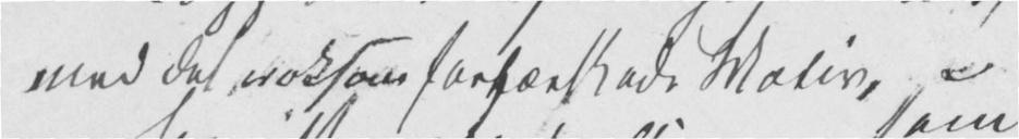med det noksom fortærskede Motiv,
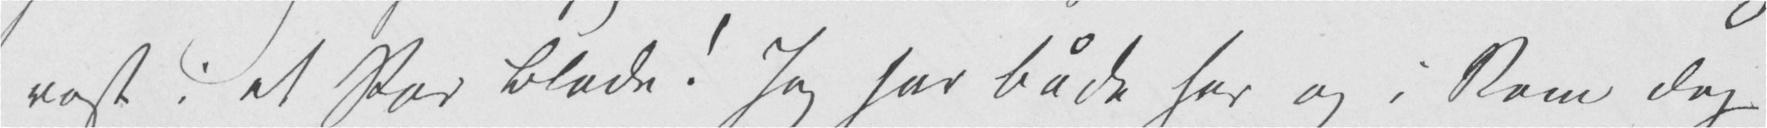rost i et Par Blade! Jeg har både her og i Rom dog
